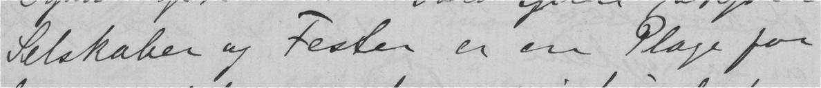Selskaber og Fester er en Plage for
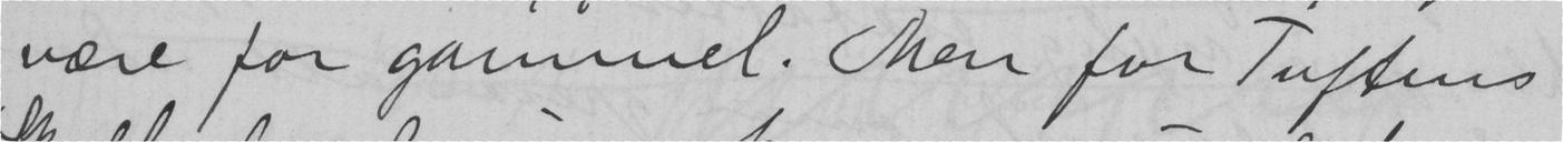være for gammel. Men for Tuftens
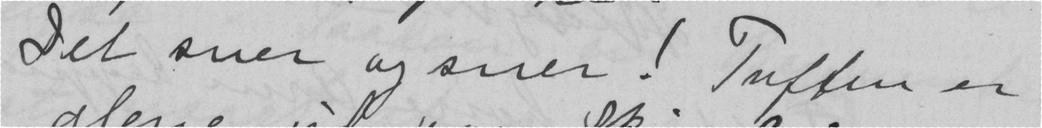Det sner og sner! Tuften er
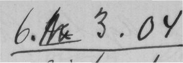6. 3.04
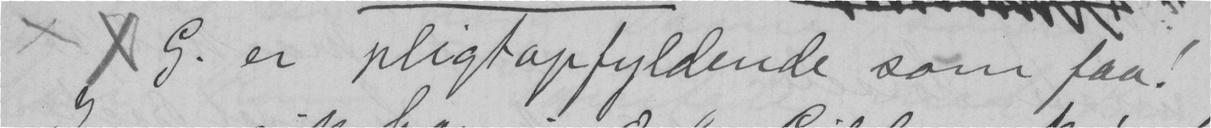x x G. er pligtopfyldende som faa!
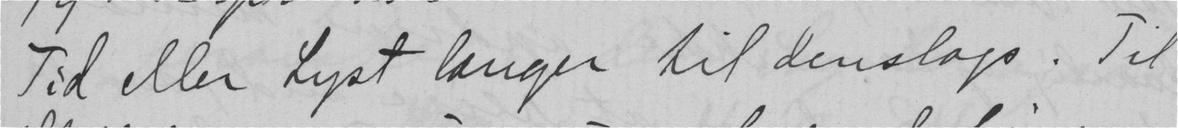Tid eller Lyst længer til denslags. Til
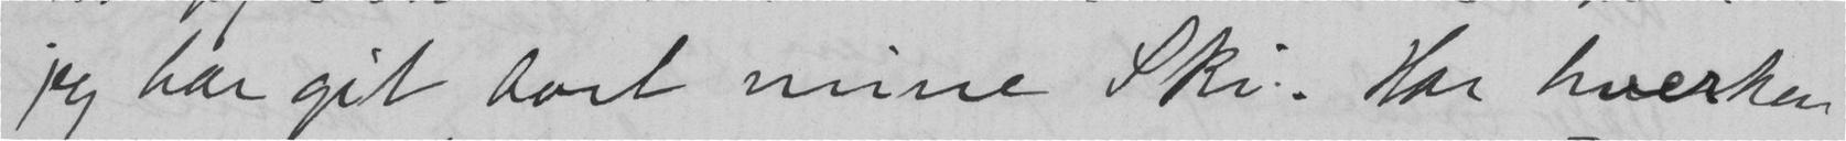Jeg har git bort mine Ski. Har hverken
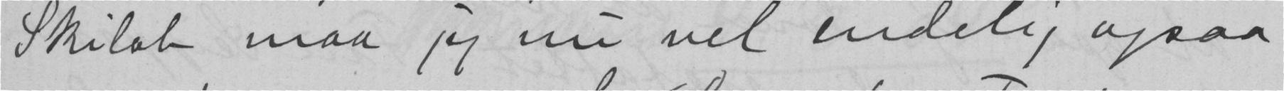Skiløb maa jeg nu vel endelig ogsaa
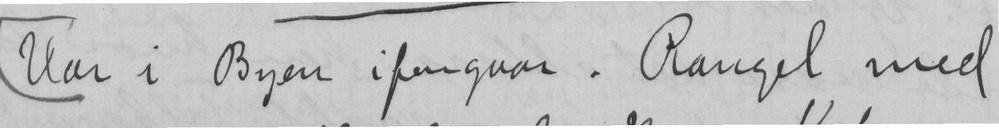Var i Byen iforgaar. Rangel med
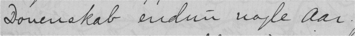Dovenskab endnu nogle Aar.
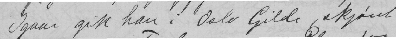Igaar gik han i Oslo Gilde, skjønt
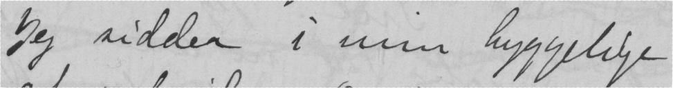Jeg sidder i min hyggelige
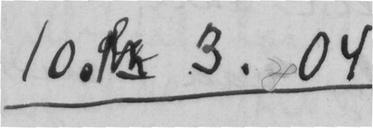10. 3.04
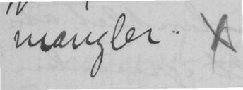mangler. x
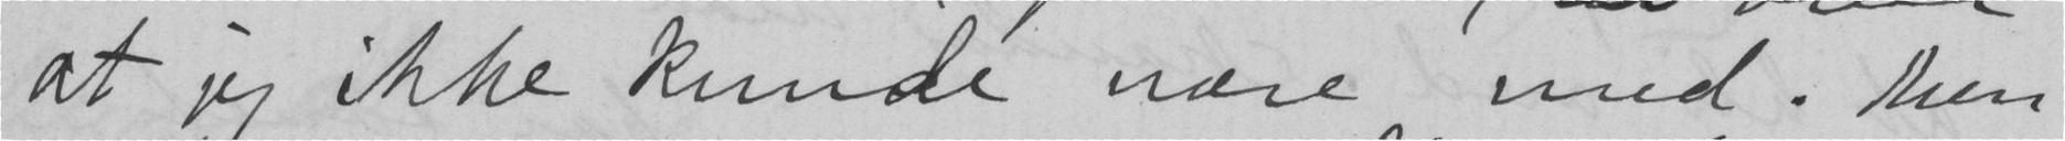at jeg ikke kunde være med. Men
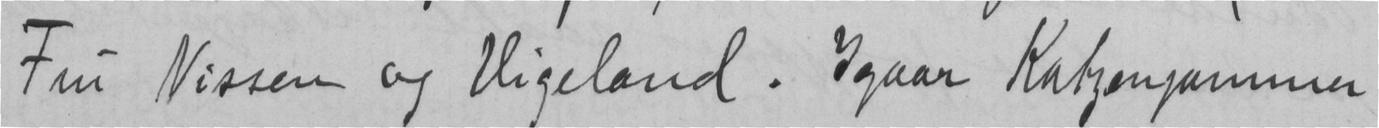Fru Nissen og Vigeland. Igaar Katzenjammer
